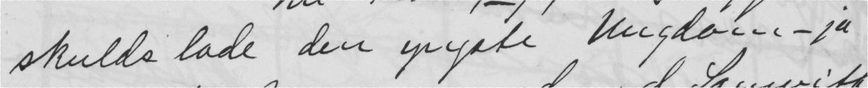skulde lade den yngste Ungdom - ja
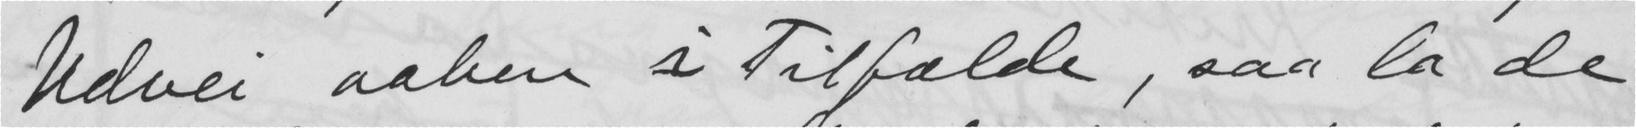Udvei aaben s Tilfælde, saa la de
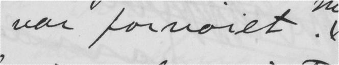var fornøiet.
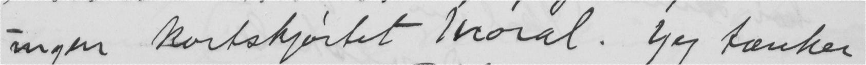ingen Kortskjørtet Moral. Jeg tænker
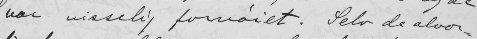var visselig fornøiet. Selv de alvor-
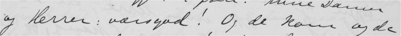og Herrer værsgod! Og de kom og de
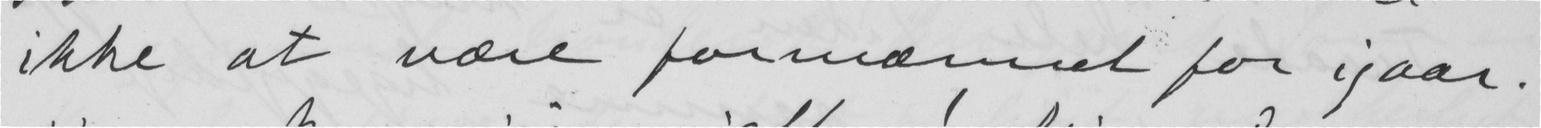ikke at være fornærmet for igaar.
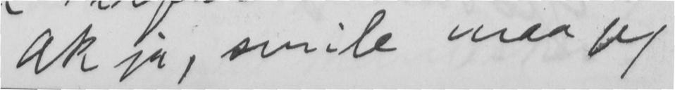Ak ja, smile maa jeg
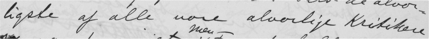ligste af alle vore alvorlige Kritikere
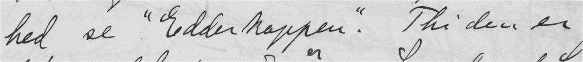hed se "Edderkoppen". Thi den er
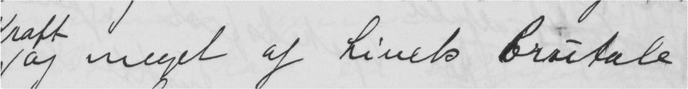og meget af Livets brutale
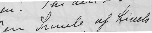en Smule af Livets
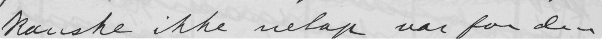kanske ikke nelop var for den
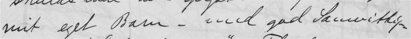mit eget Barn — med god Samvittig-
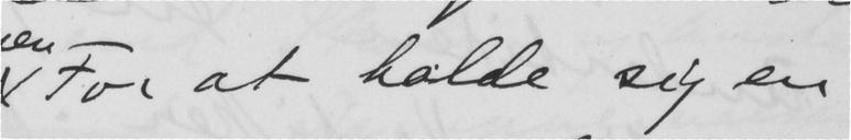For at holde sig en
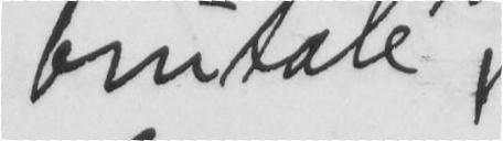brutale
